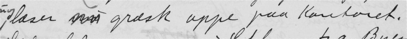læser  græsk oppe paa Kontoret.
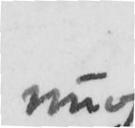nu og
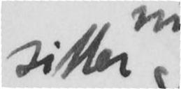sitter
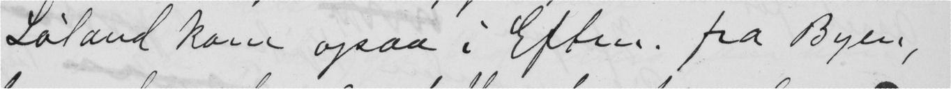Løland kom ogsaa i Eften, fra Byen,
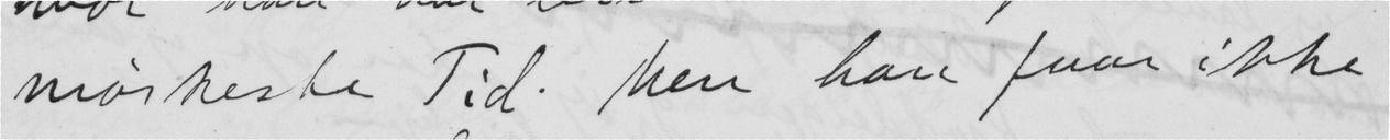mørkeste Tid. Men han faar ikke
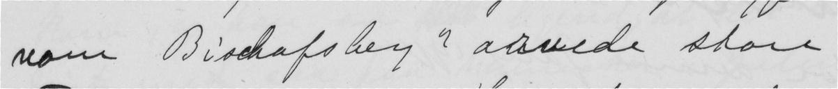vom Bischafsberg" arvede store
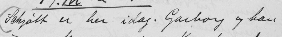Schjøtt er her idag. Garborg og han
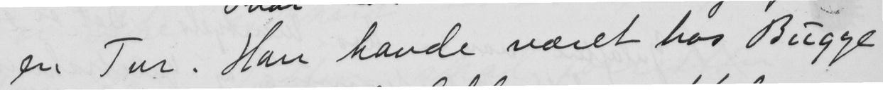en Tur. Han havde været hos Bugge
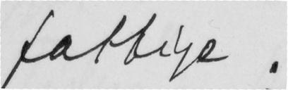fattige.
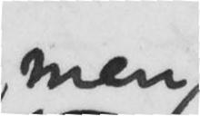men
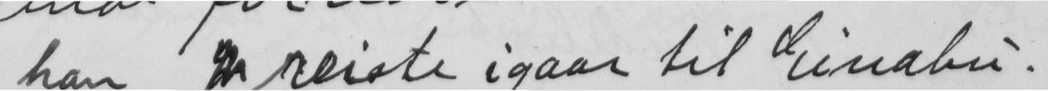han  reiste igaar til Einabu.
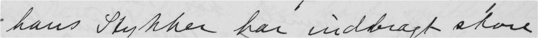hans Stykker har indbragt store
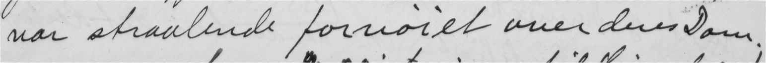var straalende fornøiet over dens Dom;
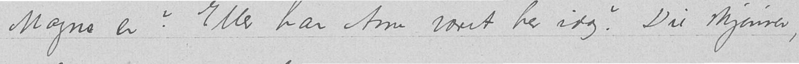Magne er? Eller har Arne vært her idag? Du skjønner,
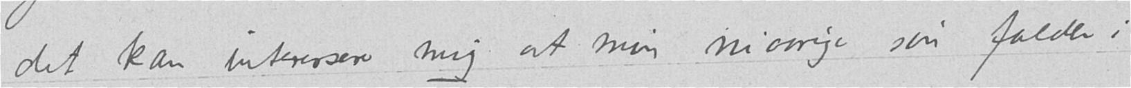det kan interessere mig at min niaarige søn falder i
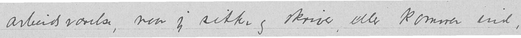arbeidsværelse, naar jeg sitter og skriver, eller kommer ind,
